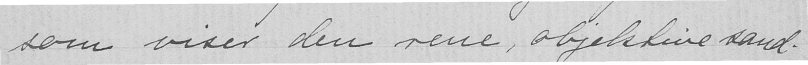som viser den rene, objektive sand-
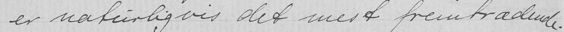er naturligvis det mest fremtrædende.
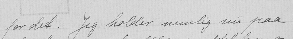for det. Jeg holder nemlig nu paa
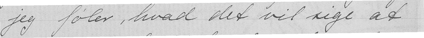jeg føler, hvad det vil sige at
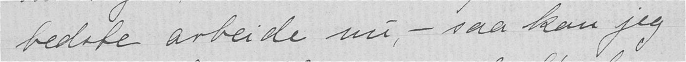bedste arbeide nu, - saa kan jeg
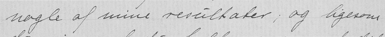nogle af mine resultater; og ligesom
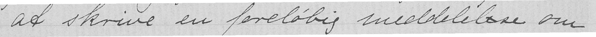at skrive en foreløbig meddelelse om
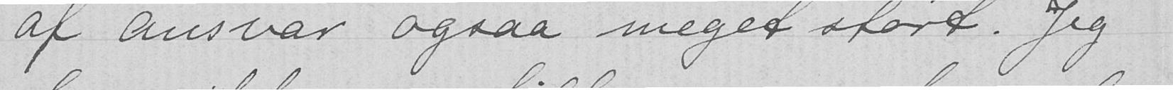af ansvar ogsaa meget stort. Jeg
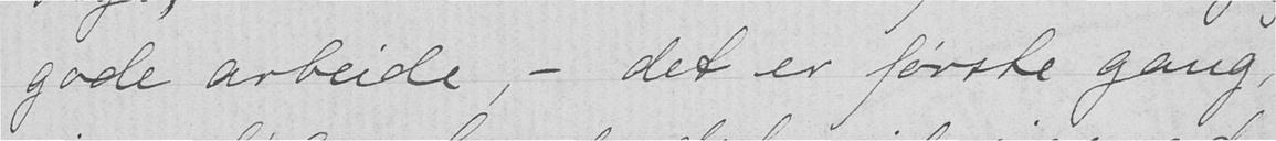gode arbeide, - det er første gang,
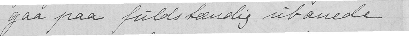gaa paa fuldstændig ubanede
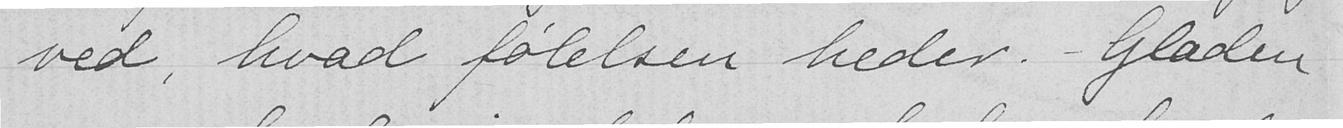ved, hvad følelsen heder. - Glæden
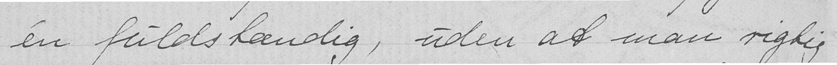en fuldstændig, uden at man rigtig
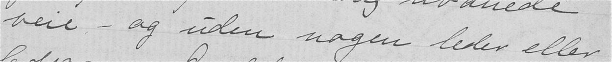veie, - og uden nogen leder eller
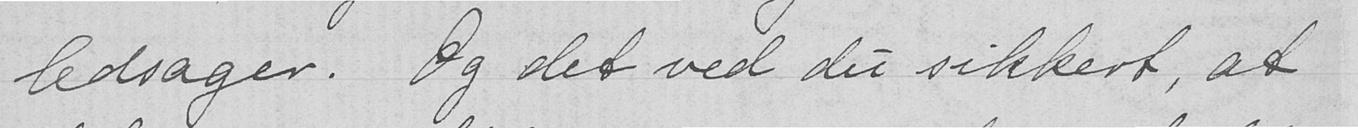ledsager. Og det ved du sikkert, at
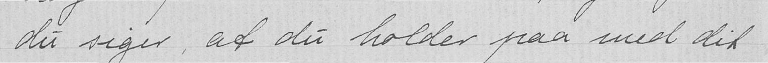du siger, at du holder paa med dit
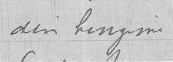din hengivne
